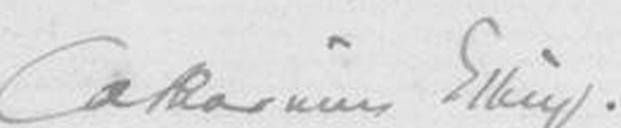Catharinus Elling
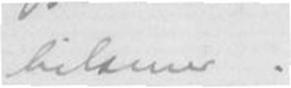hilsener.
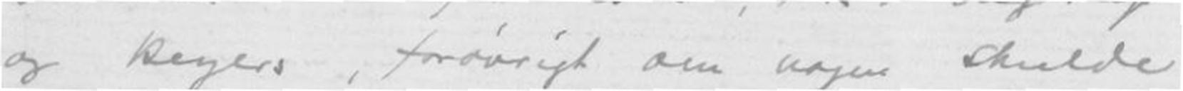og Beyers, forøvrigt om nogen skulde
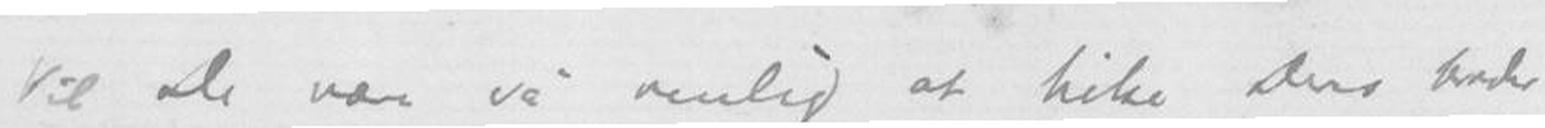Vil De være så venlig at hilse Deres broder
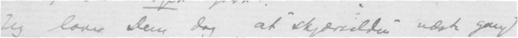Jeg love Dem dog at "skjærsilden" næste gang
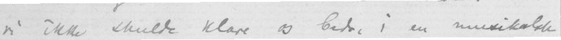vi ikke skulde klare os bedre i en musikalsk
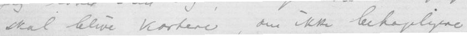skal blive kortere, om ikke behageligere,
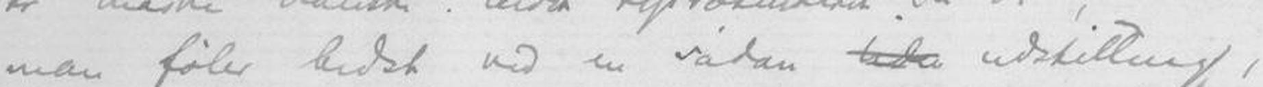man føler bedst ved en sådan Uds udstilling,
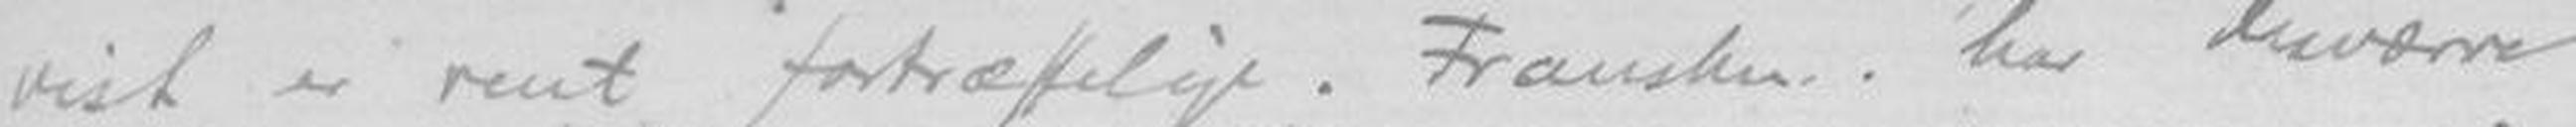vist er rent fortræffelige. Fransker har desværre
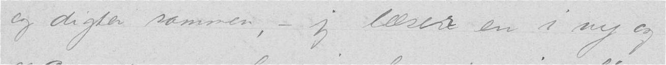og digter sammen, — jeg læser en i ny og
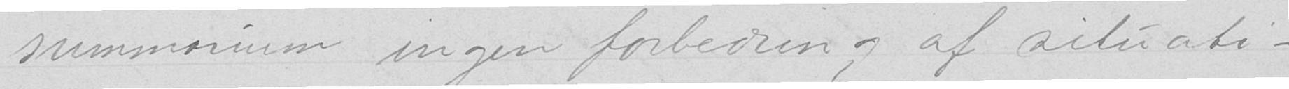summarum ingen forbedring af situati-
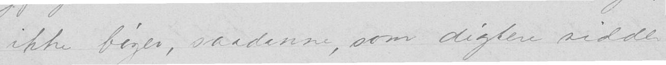ikke bøger, saadanne, som digtere sidder
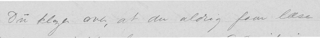Du klager over, at du aldrig faar læse —
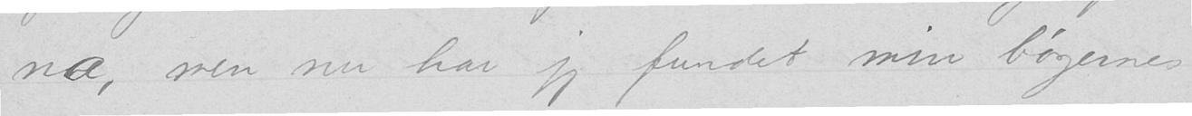ne, men nu har jeg fundet min bøgernes
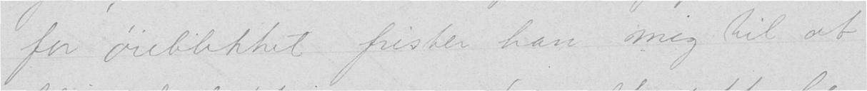for øieblikket frister han mig til at
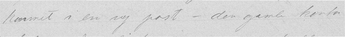kommet i en ny post — den gamle kontor-
